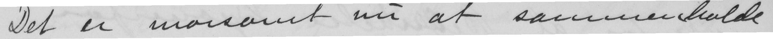Det er morsomt nu at sammenholde
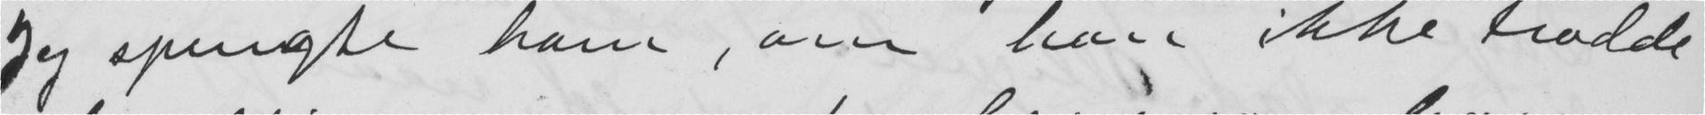Jeg spurgte ham, om han ikke trodde
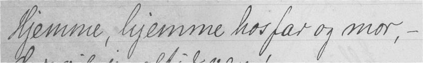Hjemme, hjemme hos far og mor, -
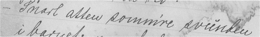- Snart atten somm're svunden
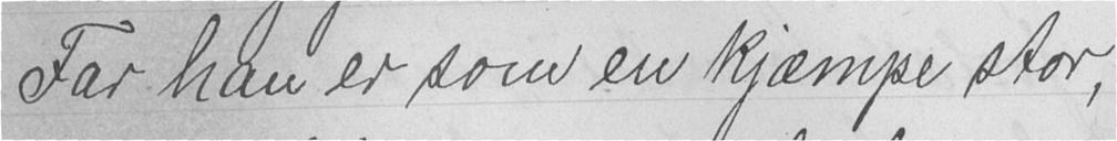Far han er som en kjæmpe stor,
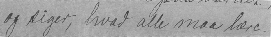og siger, hvad alle maa lære.
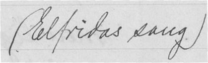(Elfridas sang.)
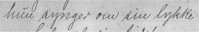hun synger om sin lykke
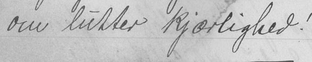om lutter kjærlighed!
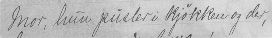Mor, hun pusler i kjøkken og der,
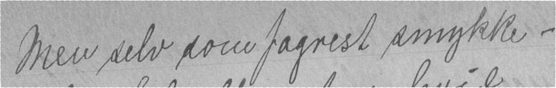Men selv som fagrest smykke -
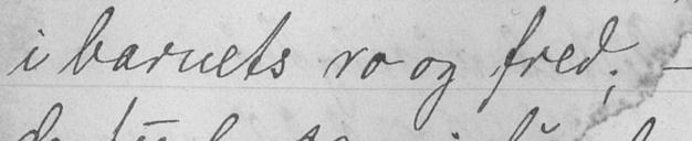i barnets ro og fred; -
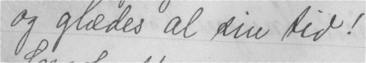og glædes al sin tid!
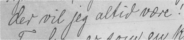der vil jeg altid være!
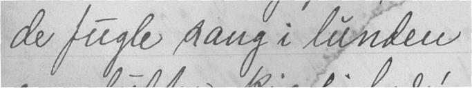de fugle sang i lunden
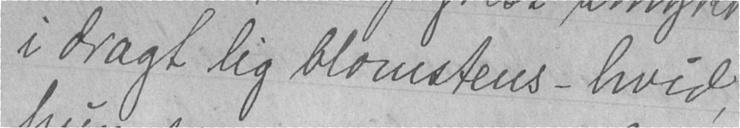i dragt lig blomstens - hvid,
